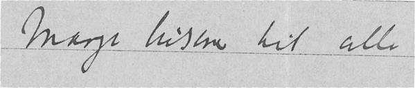Mange hilsener til alle
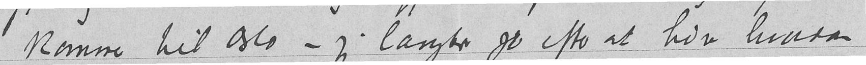kommer til Oslo – jeg længter jo efter at høre hvordan
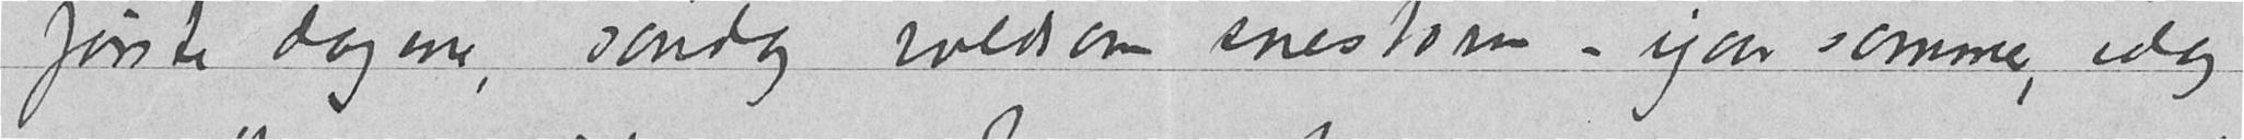første dagene, søndag voldsom snestorm – igaar sommer, idag
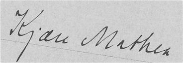Kjære Mathea
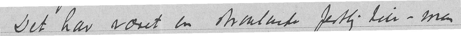Det har været en strålende festlig tur – men
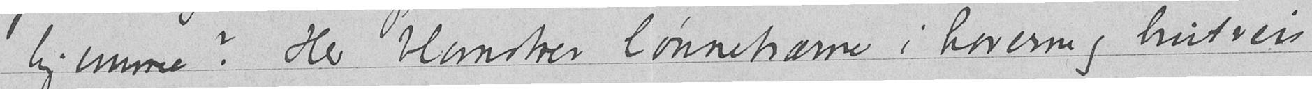hjemme?  Her blomstrer lønnetrærne i haverne og hvitveis
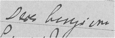Deres hengivne
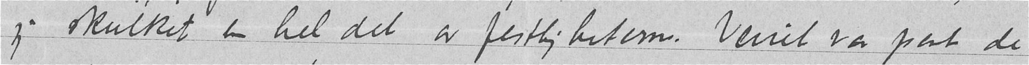jeg skulket en hel del av festligheterne. Veiret var pent de
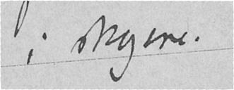i skogene.
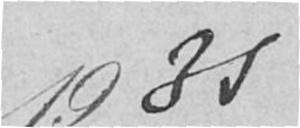1935
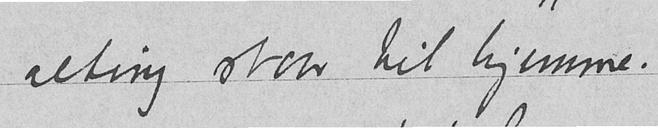alting staar til hjemme.
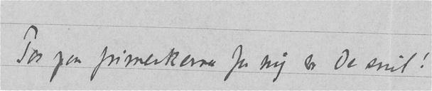Pas paa frimerkerne for mig er De snil!
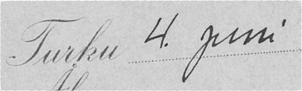Turku 4. juni
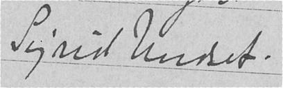Sigrid Undset.
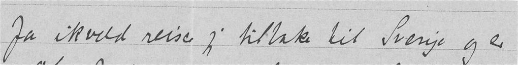Ja ikveld reiser jeg tilbake til Sverige og er
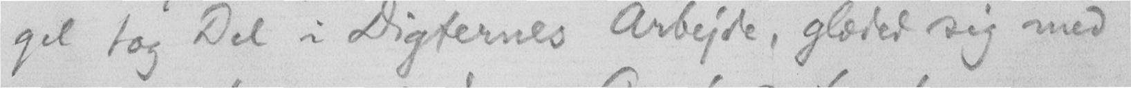gel tog Del i Digternes Arbejde, glæded sig med
